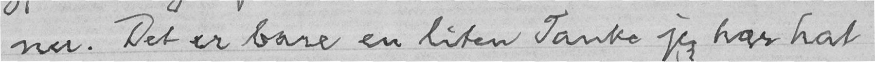nu. Det er bare en liten Tanke jeg har hat
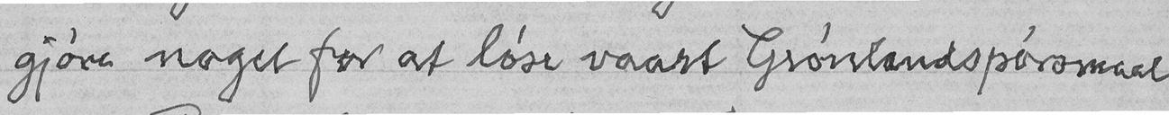gjøre noget for at løse vaart Grønlandspørsmaal
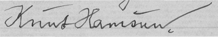Knut Hamsun.
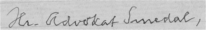Hr. Advokat Smedal,
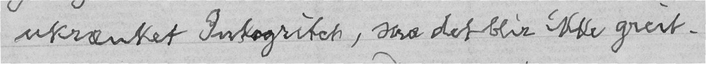ukrænket Intægritet, saa det blir ikke greit.
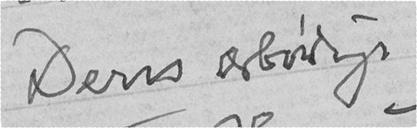Deres ærbødige
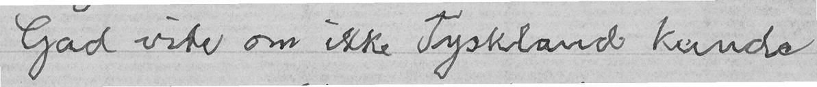Gad vite om ikke Tyskland kunde
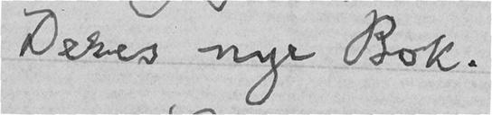Deres nye Bok.
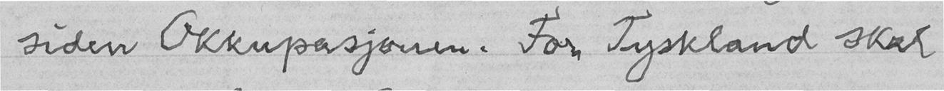siden Okkupasjonen. For Tyskland skal
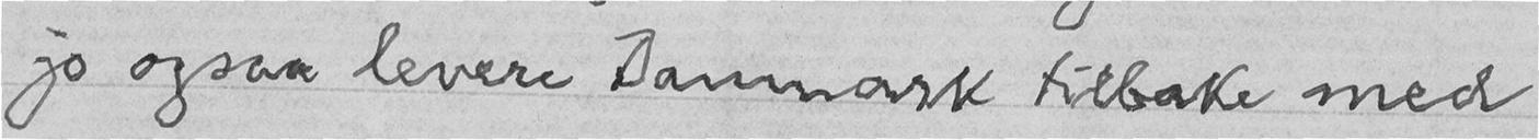jo ogsaa levere Danmark tilbake med
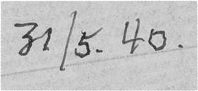31/5. 40.
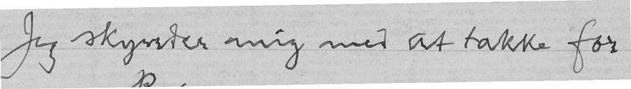Jeg skynder mig med at takke for
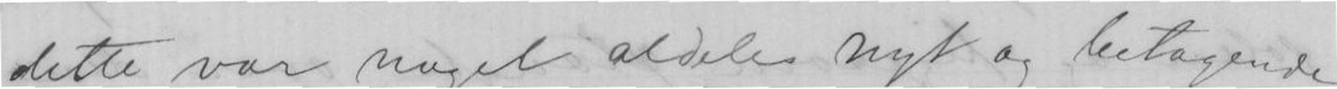dette var noget aldeles nyt og betagende
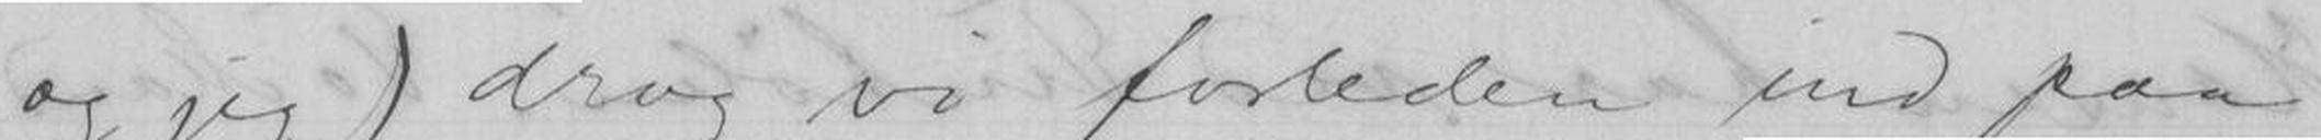og jeg) drog vi forleden ind paa
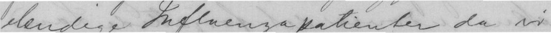elendige Influenzapatienter da vi
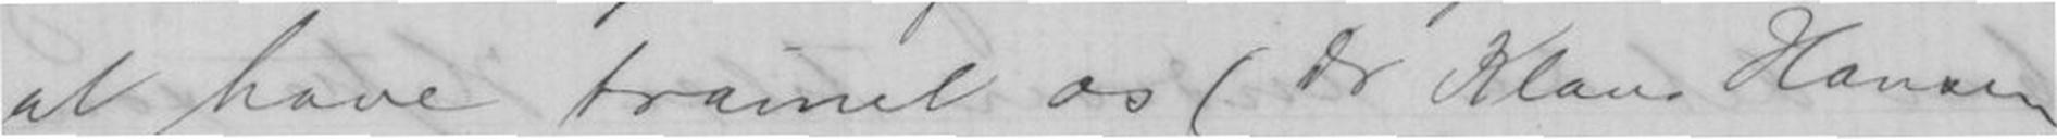at have trainet os (Dr Klaus Hansen
